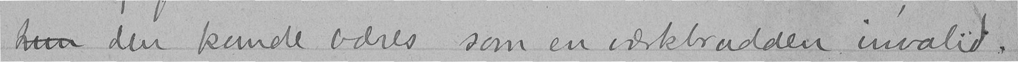hun den kunde bæres som en værkbrudden invalid.
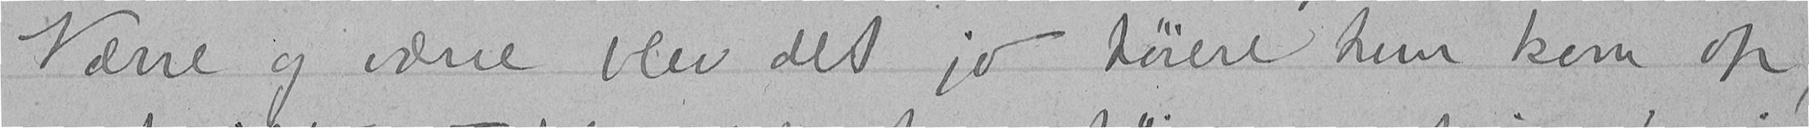Værre og værre blev det jo høiere hun kom op,
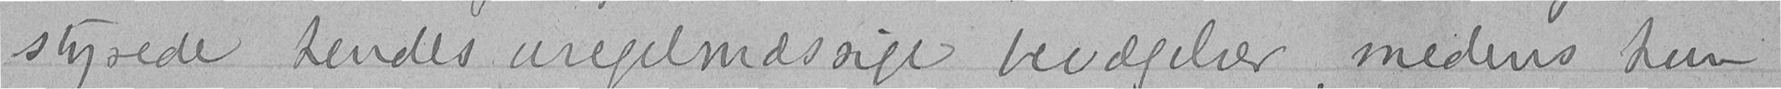styrede hendes uregelmæssige bevægelser, medens hun
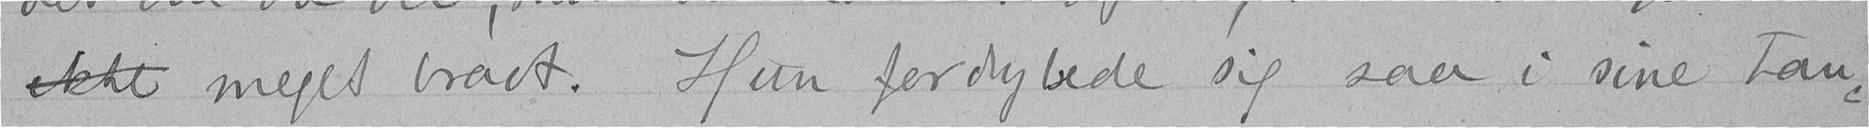ikke meget bravt. Hun fordybede sig saa i sine tan-
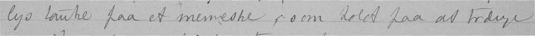lys tanke paa et menneske, som holdt paa at trænge
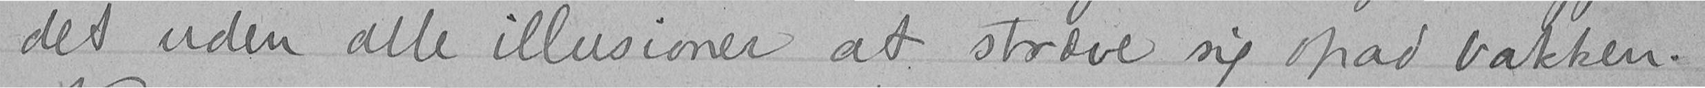det uden alle illusioner at stræve sig opad bakken.
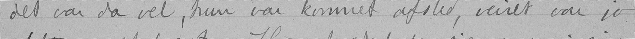det var da vel, hun var kommet afsted, veiret var jo
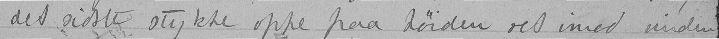det sidste stykke oppe paa høiden ret imod vinden
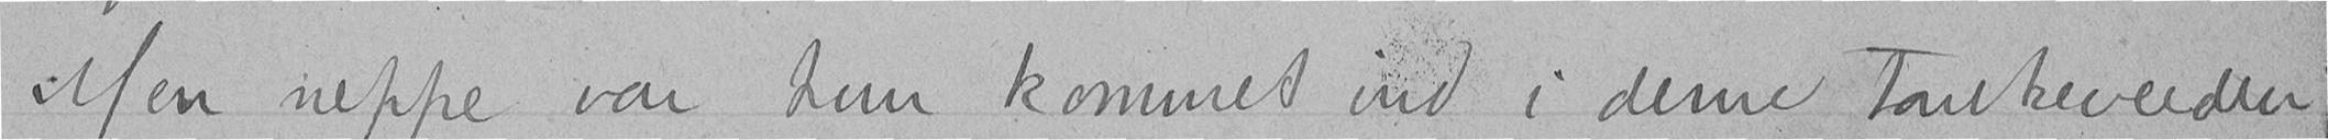Men neppe var hun kommet ind i denne tankeverden
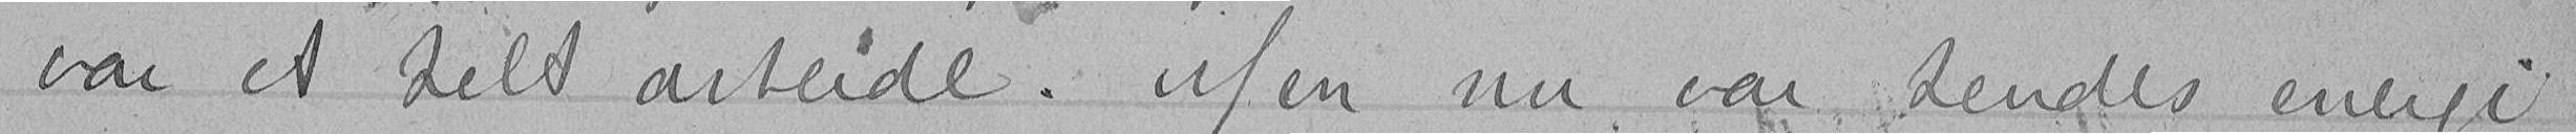var et helt arbeide. Men nu var hendes enlige
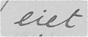eiet
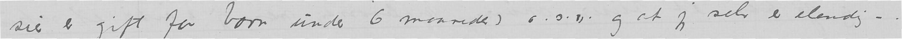sier er gift for barn under 6 maaneder) o.s.v. og at jeg selv er elendig –.
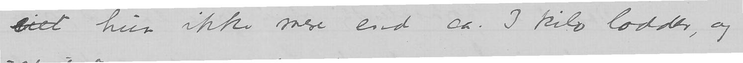eiet hun ikke mere end ca. 3 kilo lodder, og
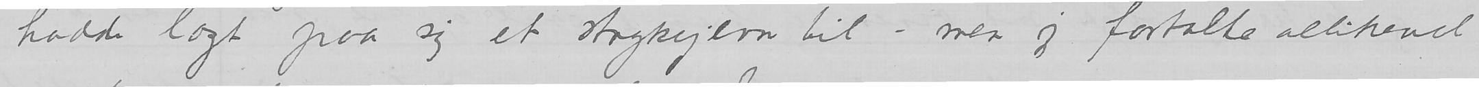hadde lagt paa sig et strykejern til – men jeg fortalte allikevel
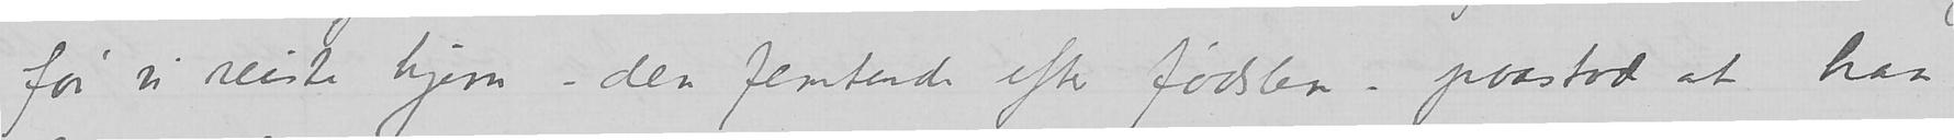før vi reiste hjem – den femtende efter fødselen – paastod at han
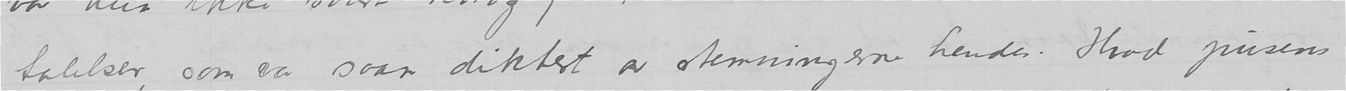talelser, som var saan diktert av stemningerne hendes. Hvad pusens
